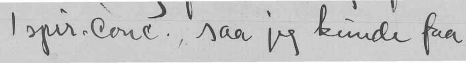1 spir-conc., saa jeg kunde faa
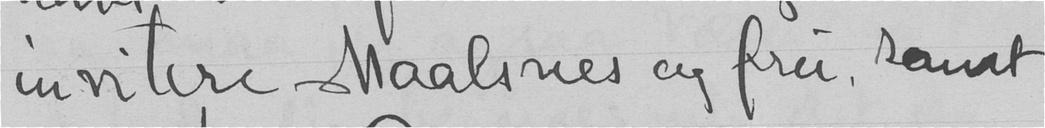invitere Maalsnes og fru, samt
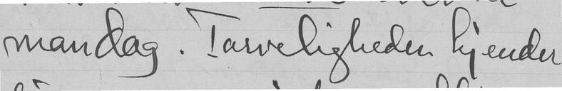mandag. Tarveligheden kjender
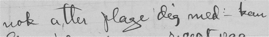nok atter plage dig med: - kan
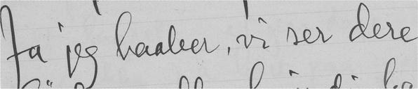Ja jeg haaber, vi ser dere
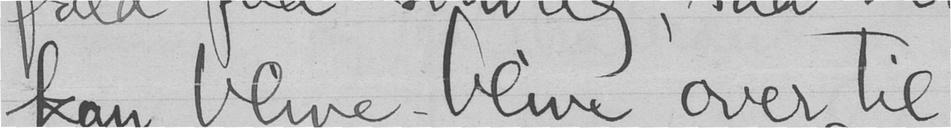kan blive - blive over til
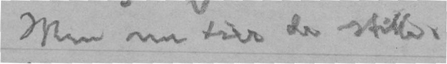Men nu tier de stille.
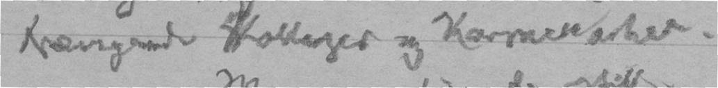trængende Kolleger og Kamerater.
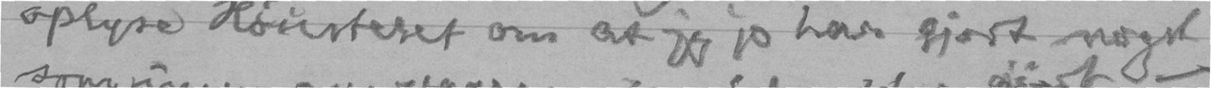oplyse Høiesteret om at jeg jo har gjort noget
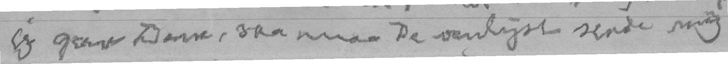jeg gav Dem, saa maa De venligst sende mig
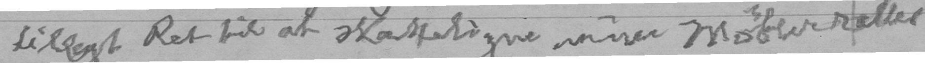tillagt Ret til at skatteligne mine Møbler r|eller
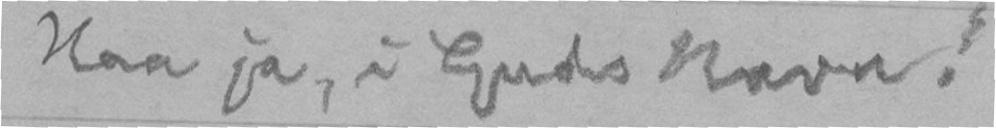Naa ja, i Guds Navn!
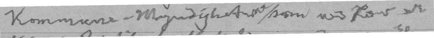Kommune-Myndigheterne /som ved Lov er
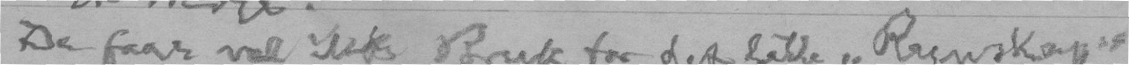De faar vel ikke Bruk for det lille "Regnskap"
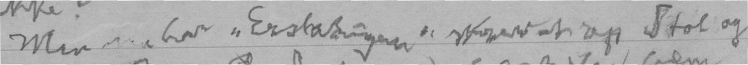Men nu har "Erstatningen" skrevet op Stol og
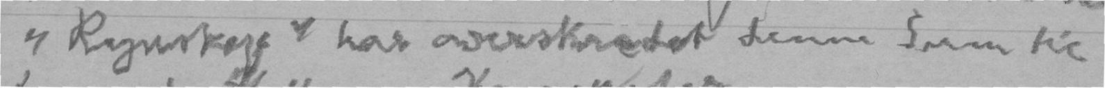"Regnskap" har overskredet denne Sum til
